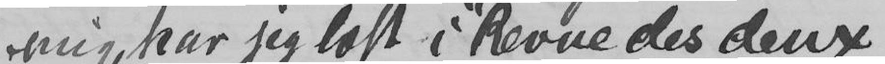mig, har jeg læst i "Revue des deux
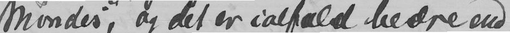Mondes", og det er ialfald bedre end
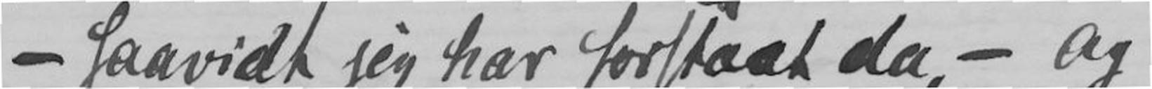-saavidt jeg har forstaat da, - og
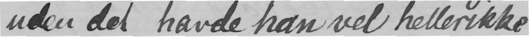uden det havde han vel heller ikke
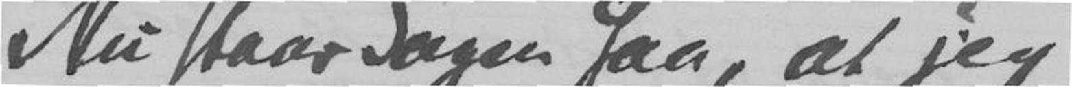Nu staar Sagen Saa, at jeg
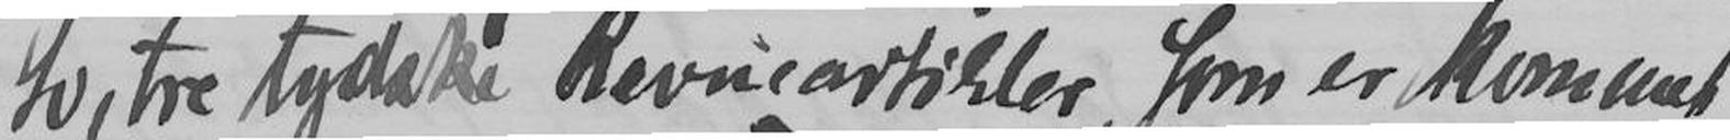to, tre tydske Revueartikler, som er kommet
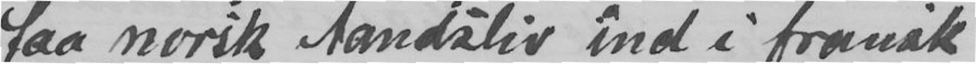faa norsk Aandsliv ind i fransk
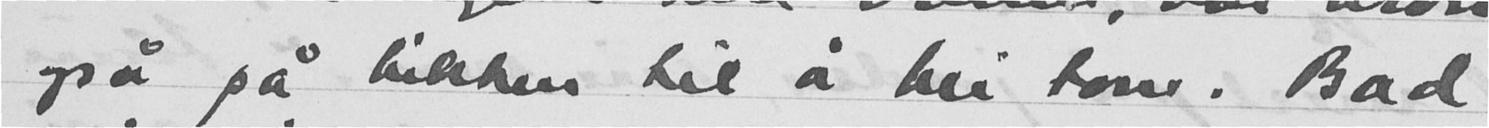også på bikken til å bli tom. Bad
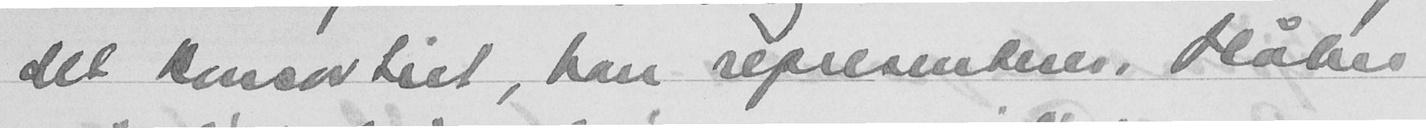det konsernet, han representerer. Håber
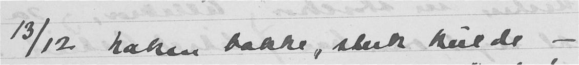13/12 Naken bakke, sterk kulde -
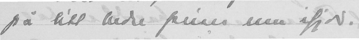på litt bedre priser enn ifjor.
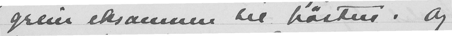greier eksamnen til høsten. Og
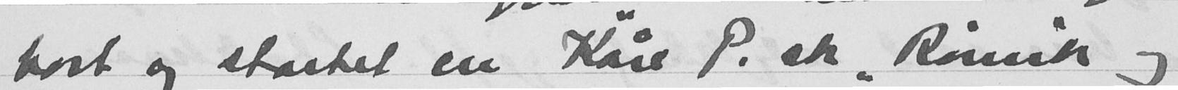bort og startet en Kåre P. sk "Reinert og
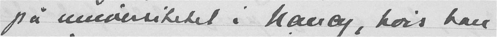på universitetet i Nancy, hvis han
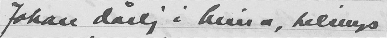Johan dårlig i beina, tilsengs
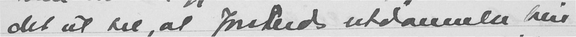det ut til, at Jonfrids utdannelse blir
